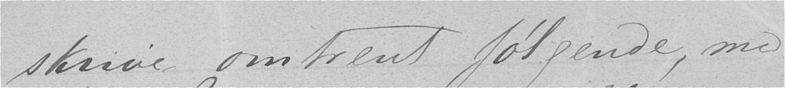skrive omtrent følgende, med
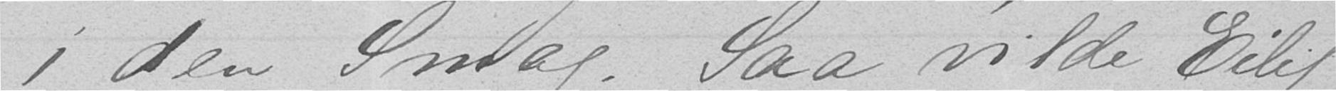i den Smag. Saa vilde Eilif
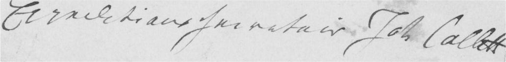Expeditionssecretair Joh Collett
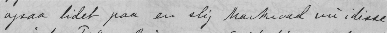ogsaa lidet paa en slig Marknad nu i disse
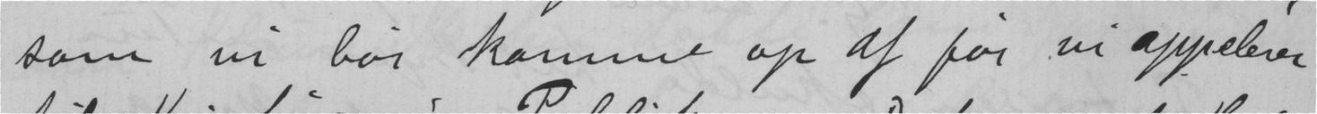som vi bør komme op af før vi appelerer
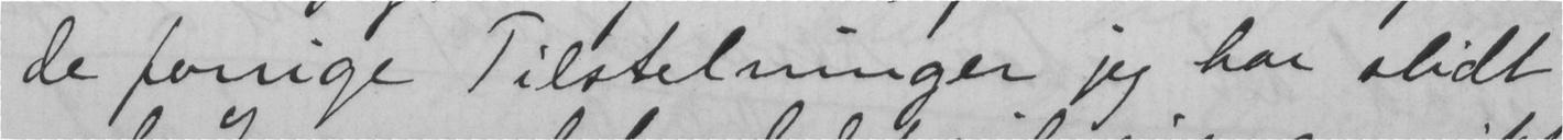de forrige Tilstelninger jeg har slidt
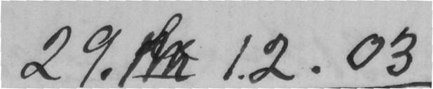29.12.03
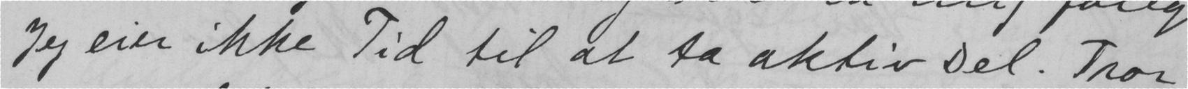Jeg eier ikke Tid til at ta aktiv Del. Tror
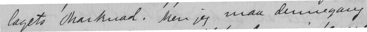lagets Marknad. Men jeg maa dennegang
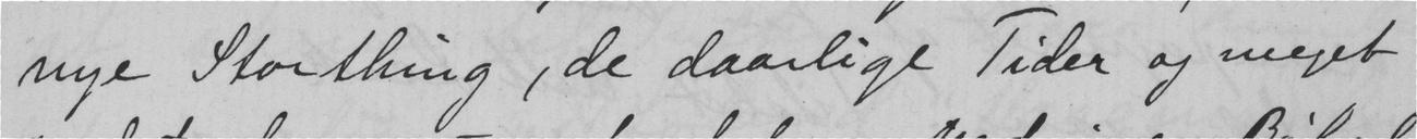nye Storthing, de daarlige Tider og meget
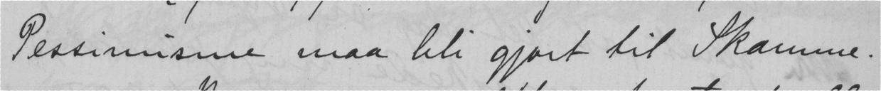Pessimisme maa bli gjort til Skamme.
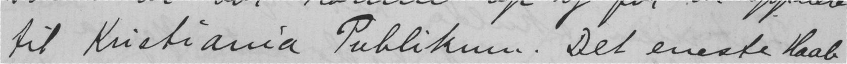til Kristiania Publikum. Det eneste Haab
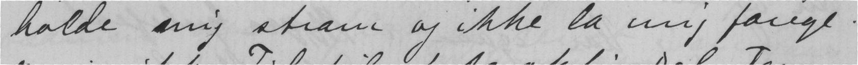holde mig stram og ikke la mig farge.
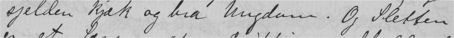sjelden kjæk og bra Ungdom. Og Sletten
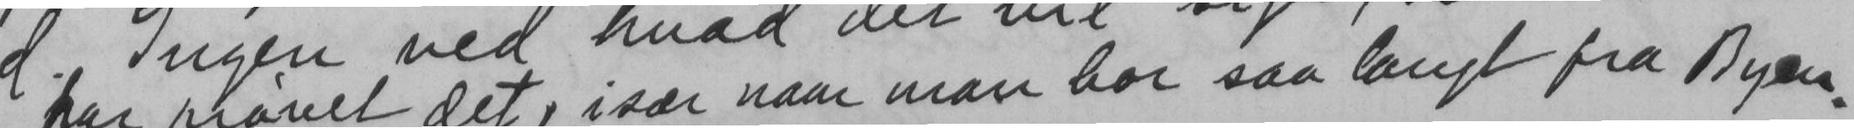par prøvet det, især naar man bor saa langt fra Byen.
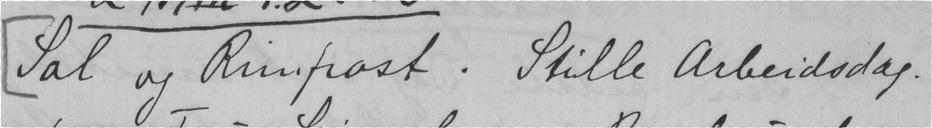Sol og Rimrost. Stille arbeidsdag.
